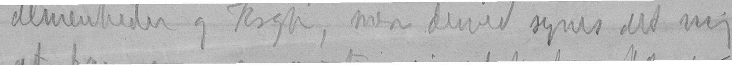almenheden og Krogh, men derved synes det mig
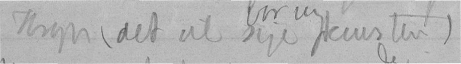Krogh (det vil sige kunsten)
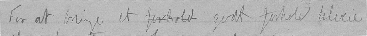For at bringe et forhold godt forhold tilveie
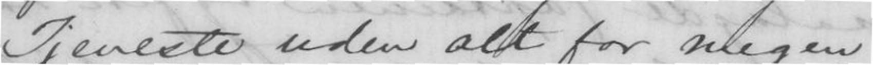Tjeneste uden alt for megen
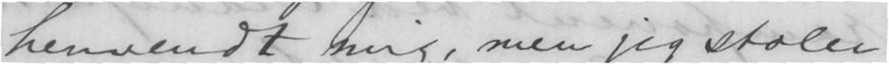henvendt mig, men jeg stoler
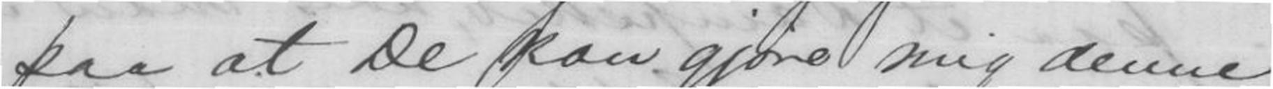paa at De kan gjøre mig denne
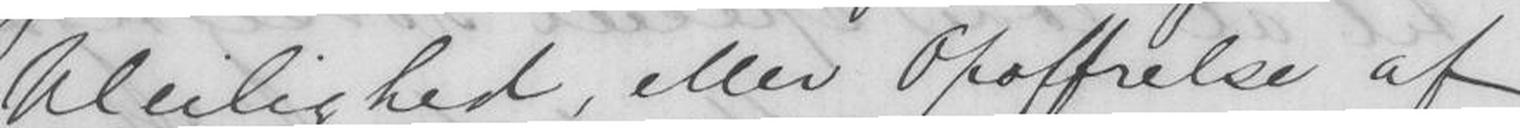Uleilighed, eller Opoffrelse af
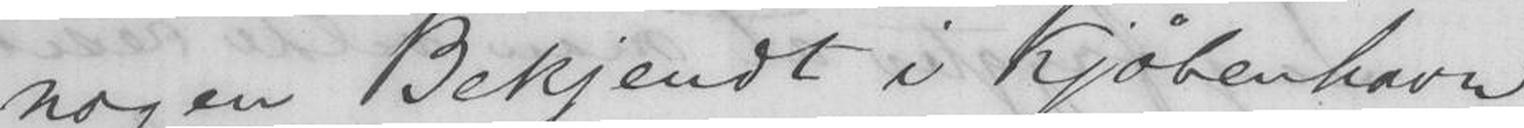nogen Bekjendt i Kjøbenhavn
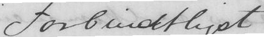Forbindtligst
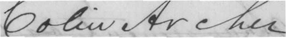Colin Archer
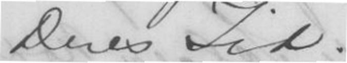Deres Tid.
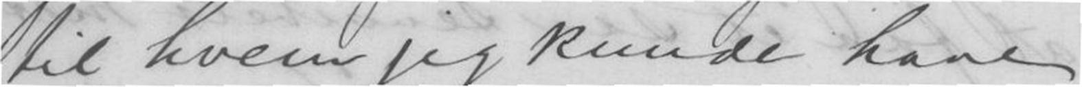til hvem jeg kunde have
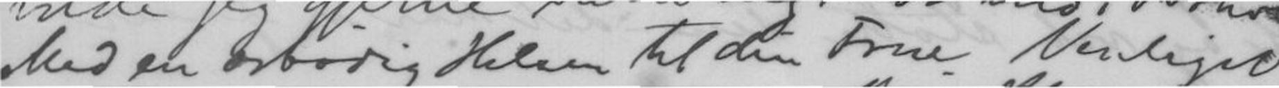Med en ærbødig Hilsen til din FrueVenligst
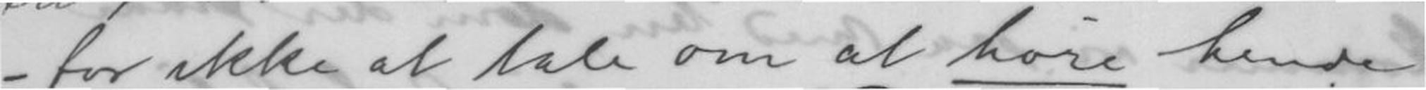- for ikke at tale om at høre hende.
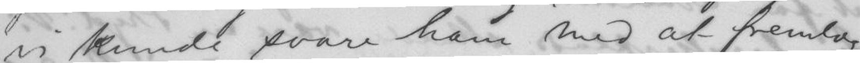vi kunde svare ham med at fremlægge
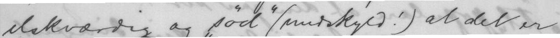elskværdig og sød (undskyld!) at det er
